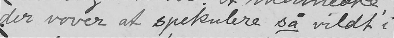der vover at spekulere så vildt i
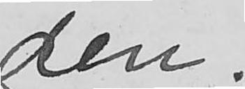den.
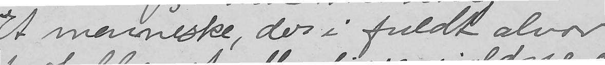Et menneske, der i fuldt alvor
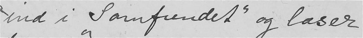ind i "Samfundet" og læser
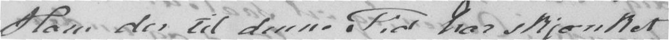Ham der til denne Tid har skjænket
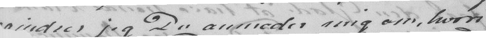indrer jeg Du anmoder mig om, hvori
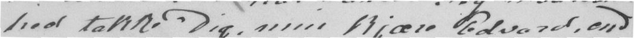hed takke Dig, min kjære Edvard, end
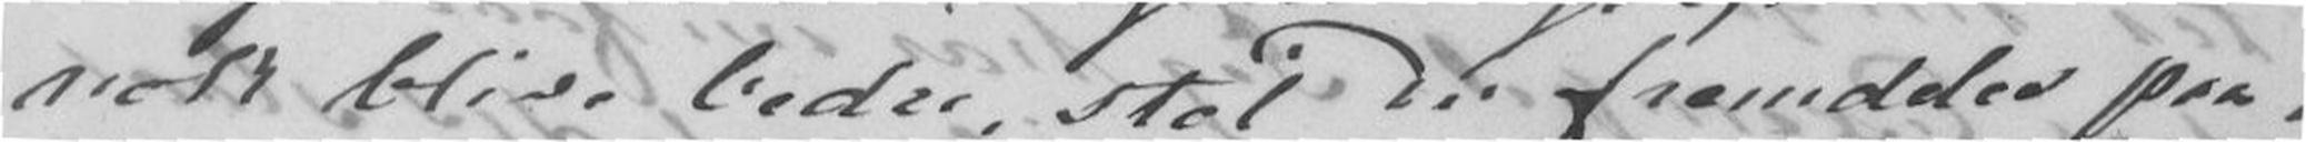nok blive bedre, stol Du fremdeles paa
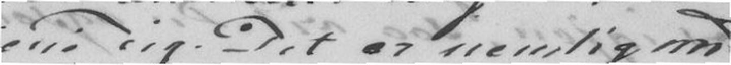tjene Dig. Det er nemlig med
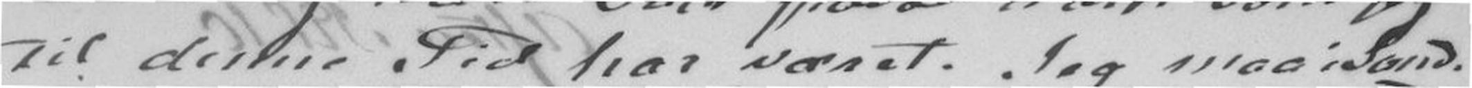til denne Tid har været. Jeg maa isand-
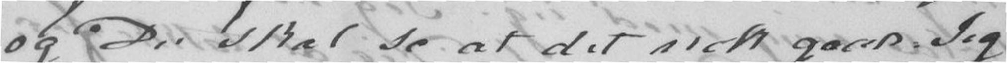og Du skal se at det nok gaar. Jeg
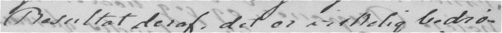Resultat deraf, det er virkelig bedrø-
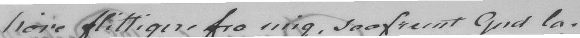høre flittigere fra mig, saafremt Gud la-
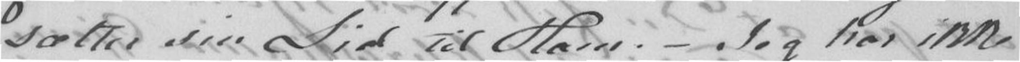sætter sin Lid til Ham. - Jeg har ikke
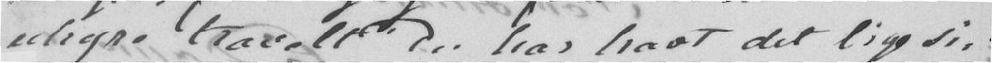uhyre travelt Du har havt det lige si-
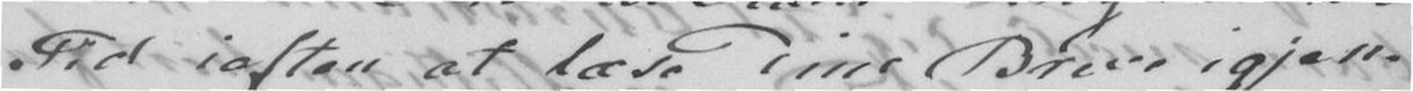Tid iaften at læse Dine Breve igjen-
